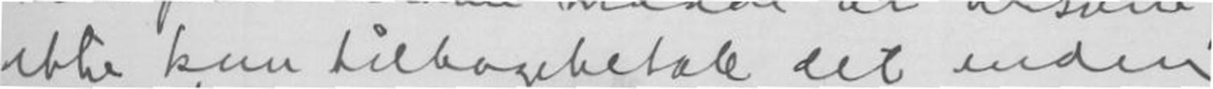ikke kan tilbagebetale det inden
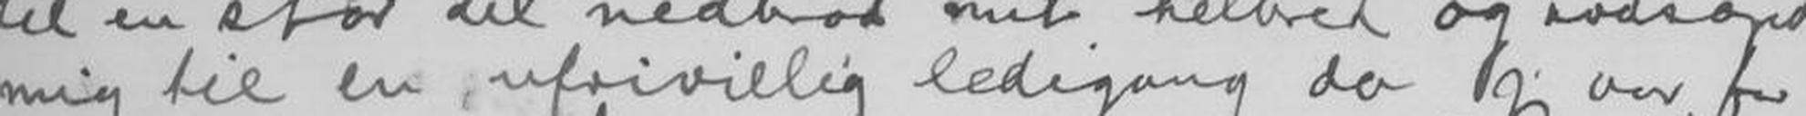mig til en ufrivillig ledigang da jeg var for
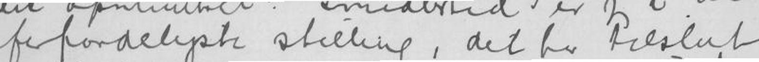forfærdeligste stilling, det har tilslut
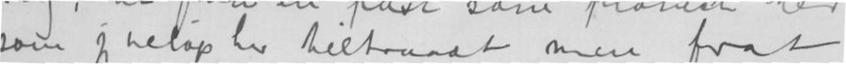som jeg netop har tiltraadt men forat
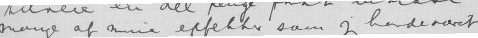mange af mine effekter her som jeg havde været
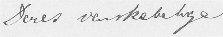Deres venskabelige
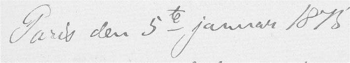Paris den 5te januar 1875.
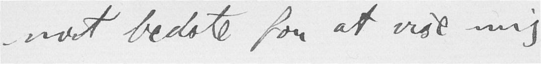mit bedste for at vise mig
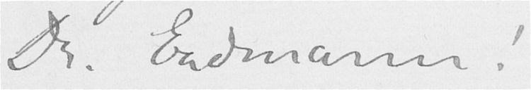Dr. Erdmann!
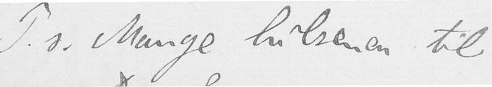P. s. Mange hilsener til
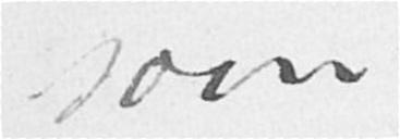som
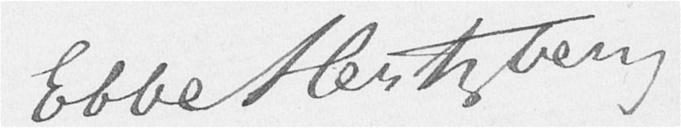Ebbe Hertzberg
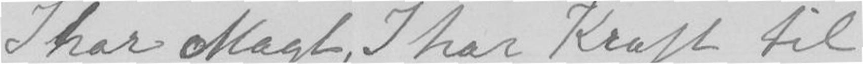I har Magt, I har Kraft til
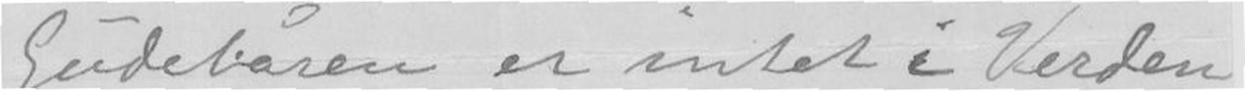Gudebåren er intet i Verden
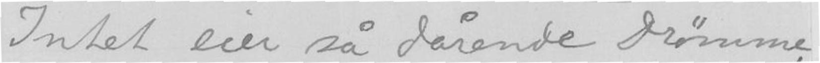Intet eier så dårende Drømme, -
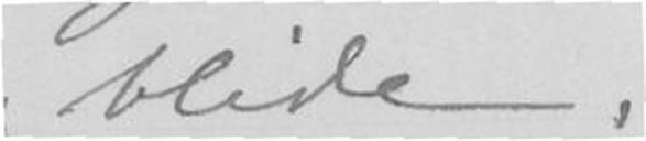blide.
